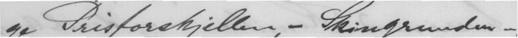ge Prisforskjellen, - Skingrunden -
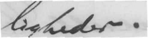ligheder.
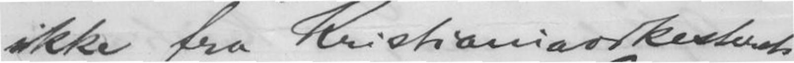ikke fra Kristianiaorkesterets
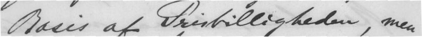Basis af Prisbilligheden, men
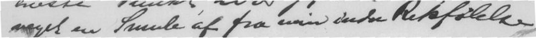veget en Smule af fra min indre Retfølelse.
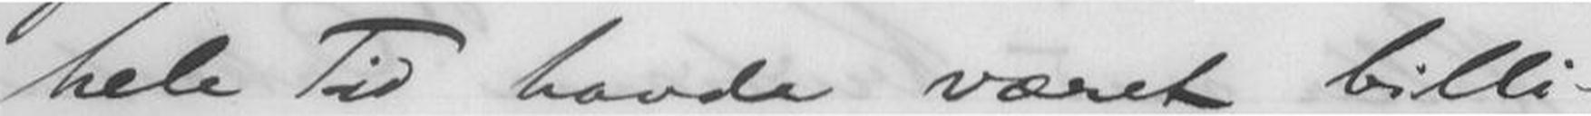hele Tid havde været billi-
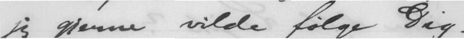jeg gjerne vilde følge Dig.
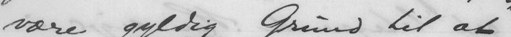være gyldig Grund til at
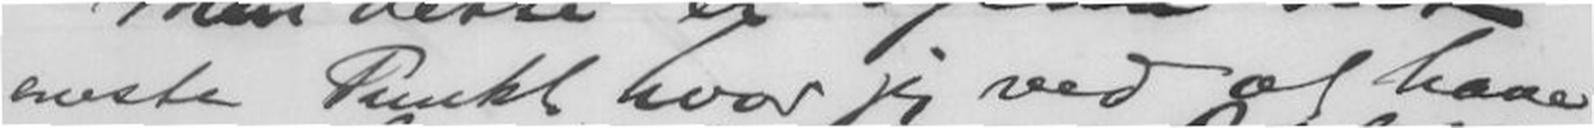eneste Punkt, hvor jeg ved at have
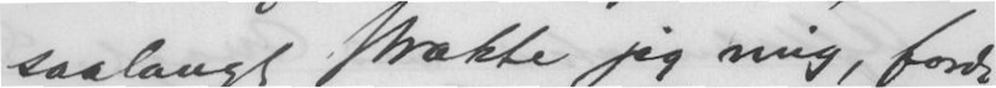saalangt strakte jeg mig, fordi
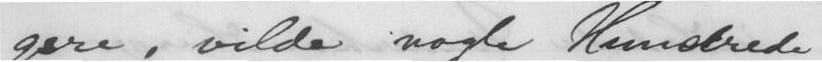gere, vilde nogle Hundrede
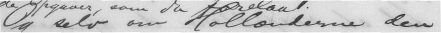Og selv om Hollænderne den
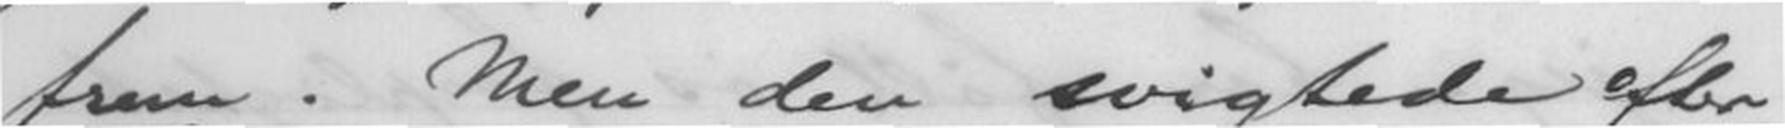frem. Men den svigtede efter
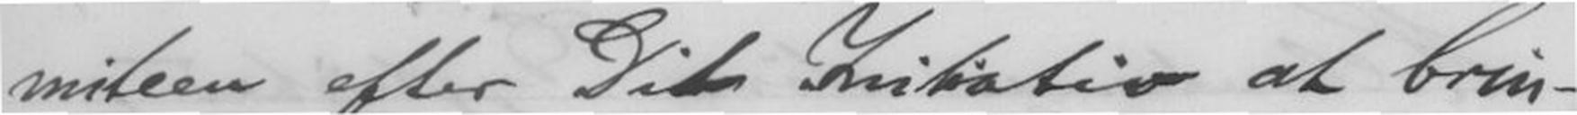miteen efter Dit Initiativ at brin-
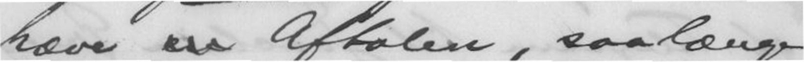hæve en Aftalen, saalænge
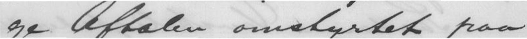ge Aftalen omstyrtet paa
